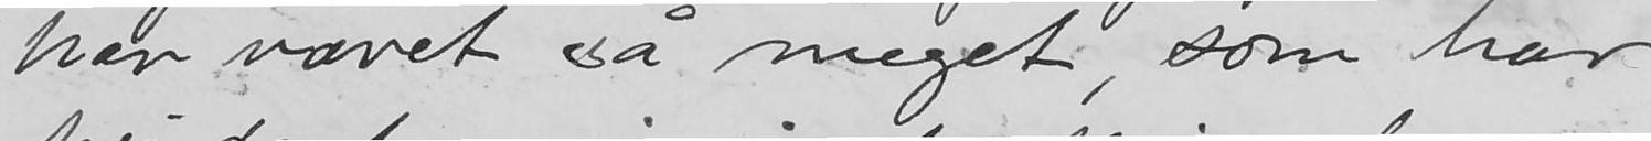har været så meget, som har
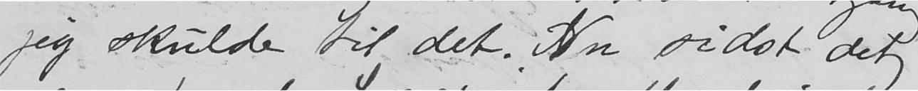jeg skulde til det. Nu sidst det
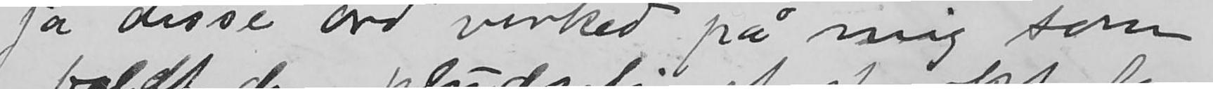Ja disse ord virked på mig som
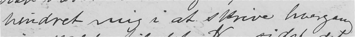hindret mig i at skrive hvergang
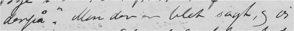derpå". Men der er blet sagt, og jeg
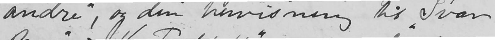andre", og din hervisning til "Ivar
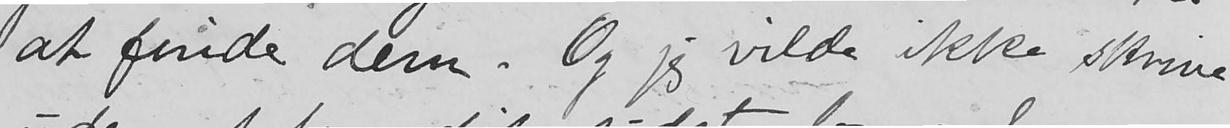at finde dem. Og jeg vilde ikke skrive
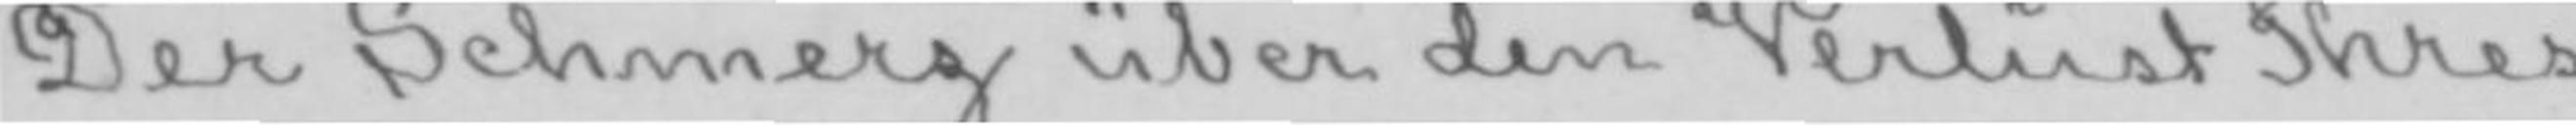Der Schmerz über den Verlust Ihres
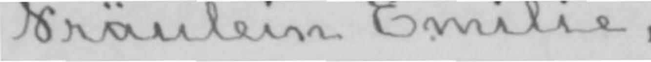Fräulein Emilie,
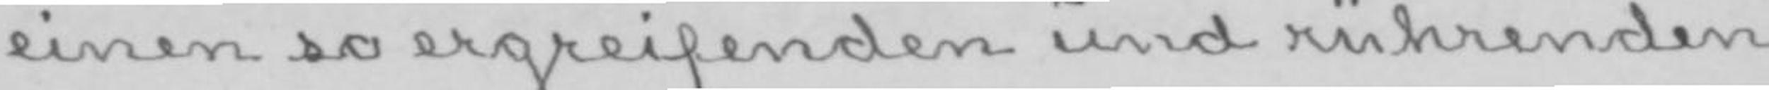einen so ergreifenden und rührenden
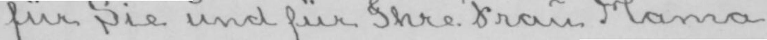für Sie und für Ihre Frau Mama
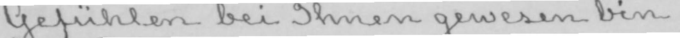Gefühlen bei Ihnen gewesen bin.
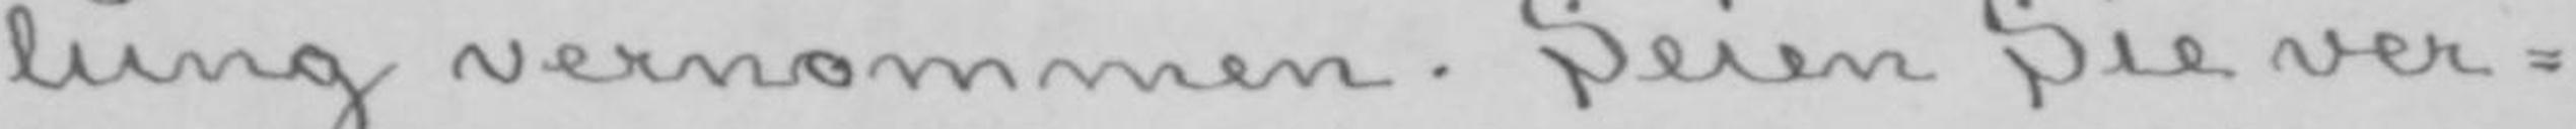lung vernommen. Seien Sie ver-
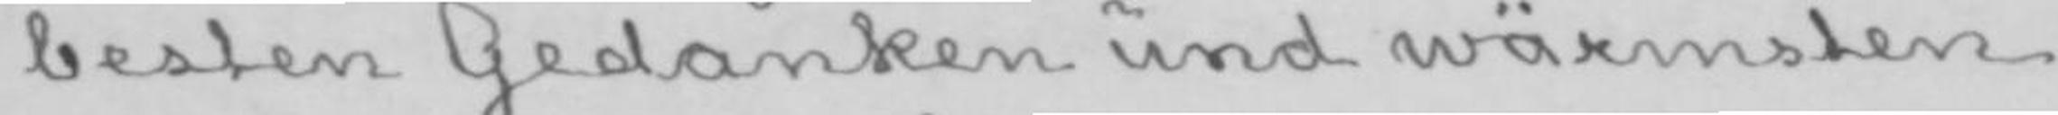besten Gedanken und wärmsten
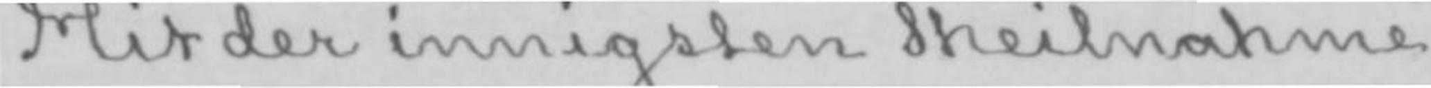Mit der innigsten Theilnahme
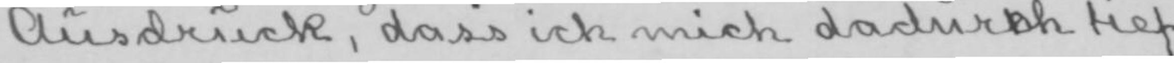Ausdruck, dass ich mich dadurch tief
